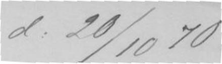d. 20/10 70
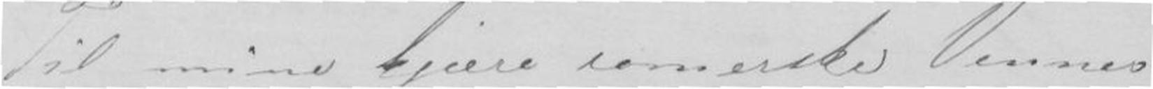Til mine kjære udmerkede Venner
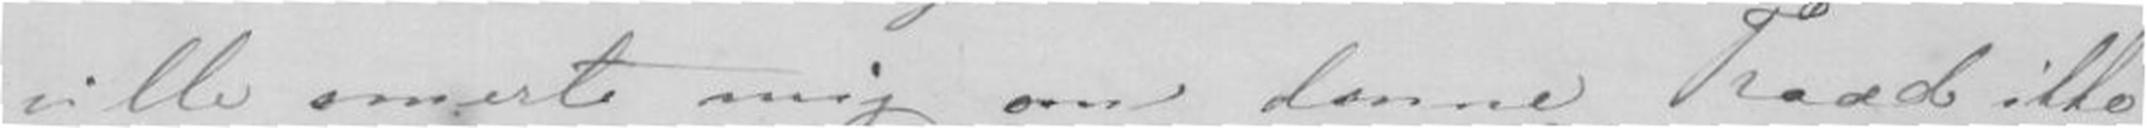ville smerte mig om denne Traad ikke
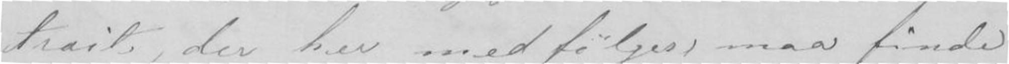trait, der her medfølger, maa finde
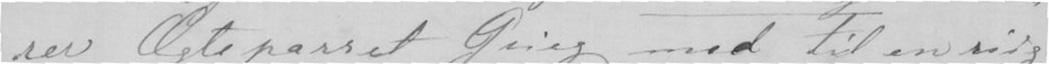rer Ægteparret Grieg med til en
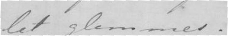let glemmes.
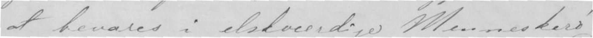at bevares i elskverdige Menneskers
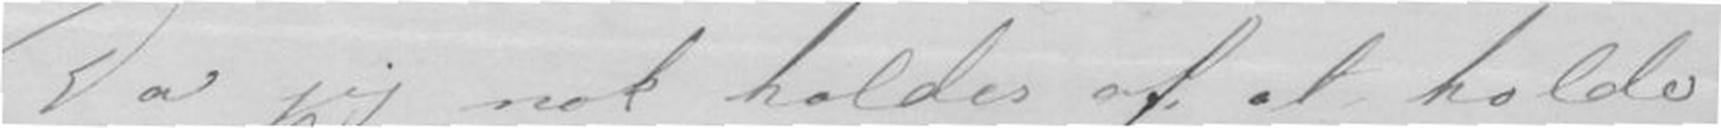Da jeg nok holder af at holde
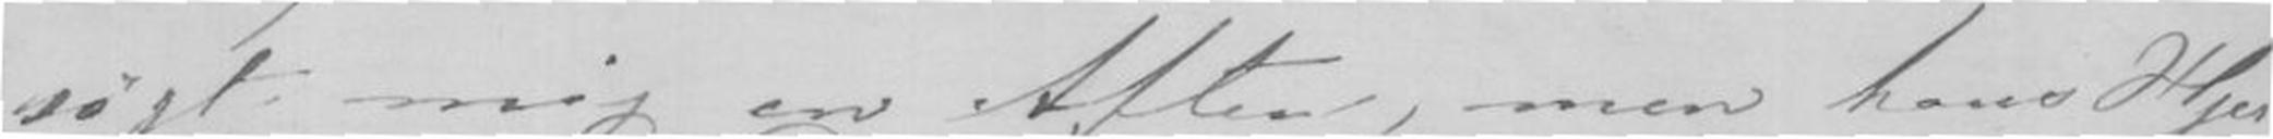søgt mig en Aften, men hans Hjer-
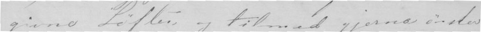givne Løfter og tilmed gjerne ønsker
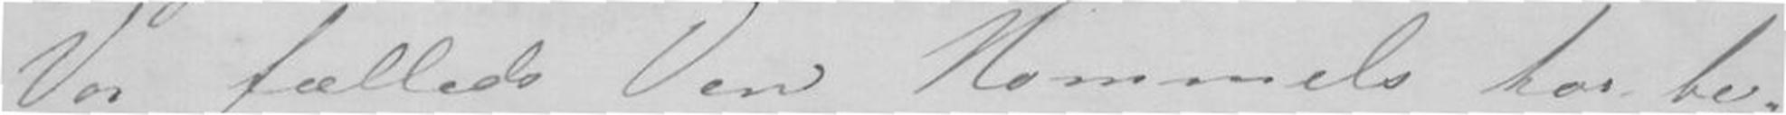Vor fælleds Ven Hommels har be-
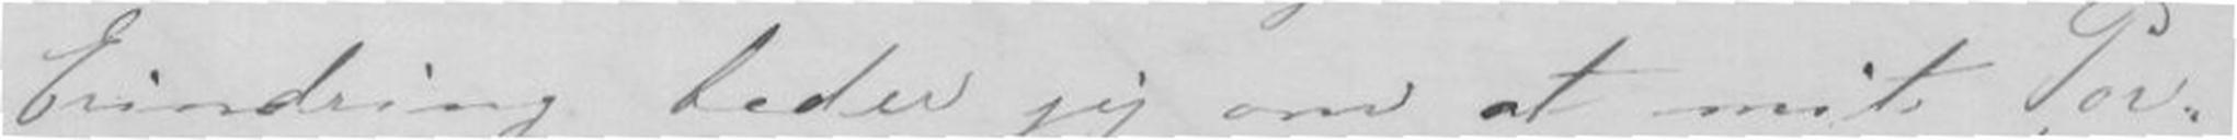Erindring beder jeg om at mit Por-
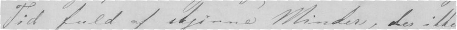Tid fuld af skjønne Minder, Les ikke
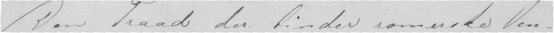Den traad der binder Ven-
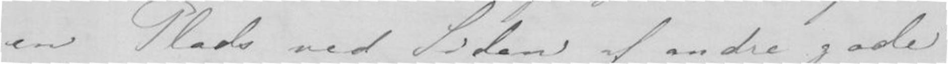en Plads ved Siden af andre gode
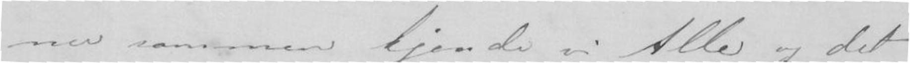ner sammen kjende vi Alle og det
